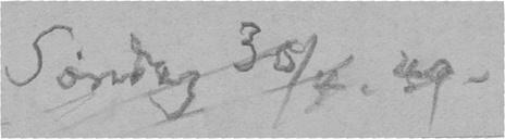Søndag 30/4. 49.
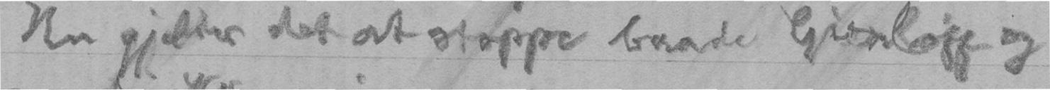Nu gjelder det at stoppe baade Gierløff og
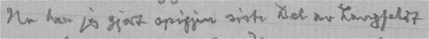Nu har jeg gjort opigjen siste Del av Langfeldt
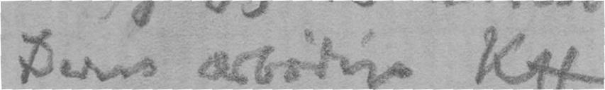Deres ærbødige KH
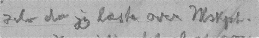selv da jeg læste over Mskpt.
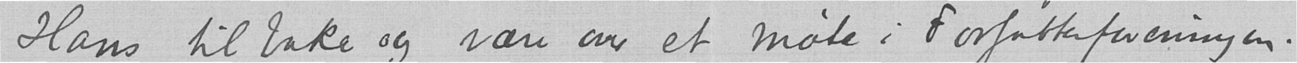Hans tilbake og være over et møte i Forfatterforeningen.
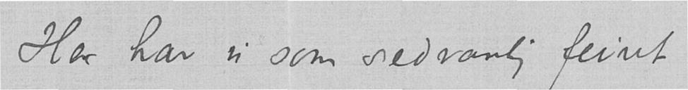Her har vi som sedvanlig feiret
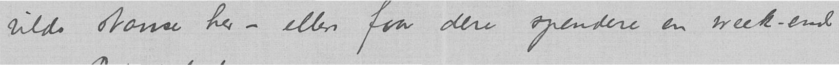vilde stanse her - ellers faar dere spendere en week-end
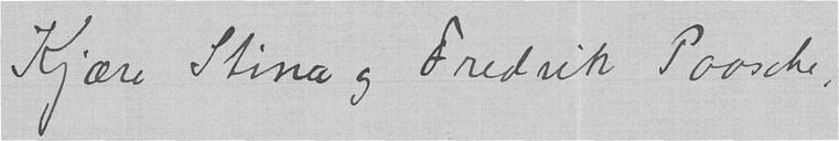Kjære Stina og Fredrik Paasche,
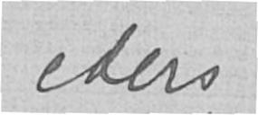eders
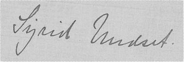Sigrid Undset.
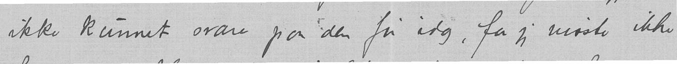ikke kunnet svare paa den før idag, for jeg visste ikke
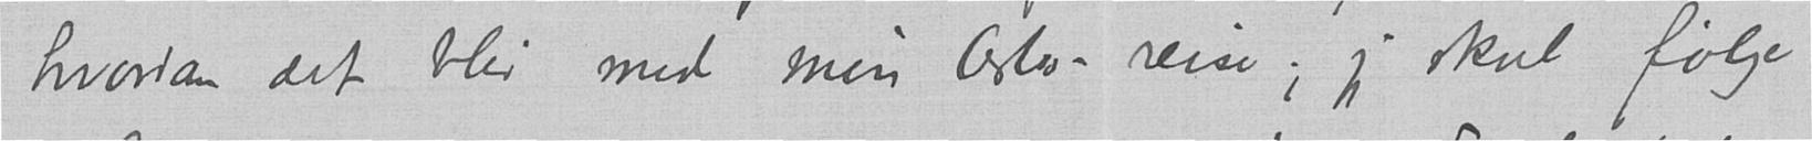hvordan det blir med min Oslo-reise; jeg skal følge
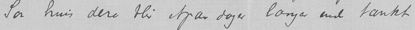Saa, hvis dere blir etpar dager længer end tænkt
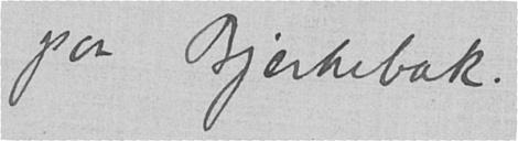paa Bjerkebæk.
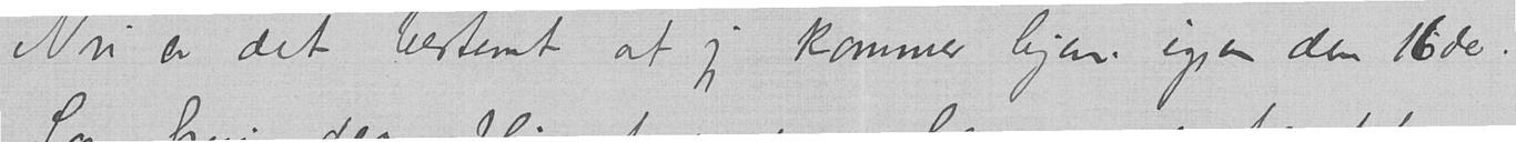Nu er det bestemt at jeg kommer hjem igjen den 16de.
